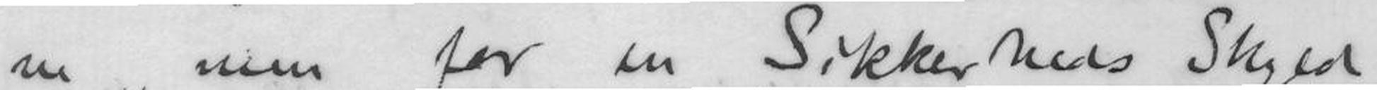ne, men for en Sikkerheds Skyld
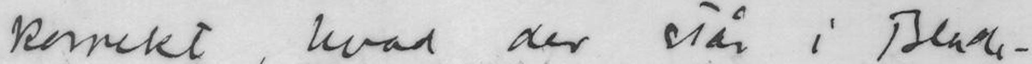korrekt, hvad der står i Blader-
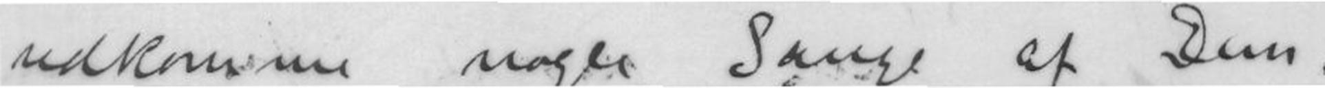udkomme nogle Sange af Dem,
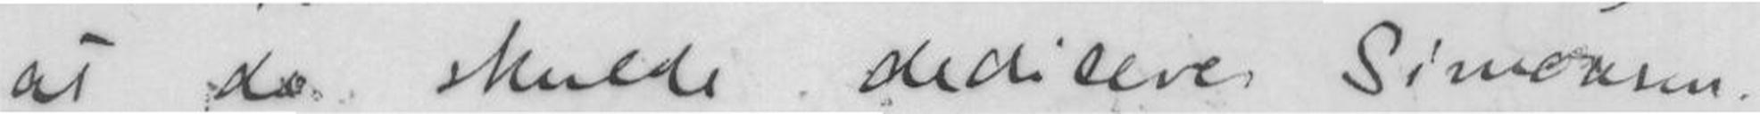at de skulde dedisere Simensen.
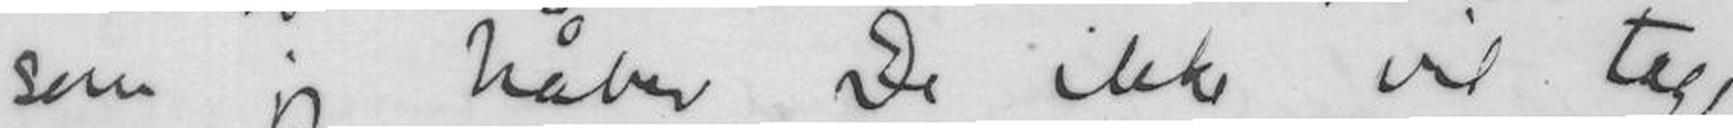som jeg håber De ikke vil tage
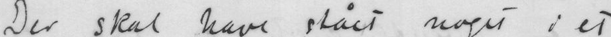Der skal have stået noget i et
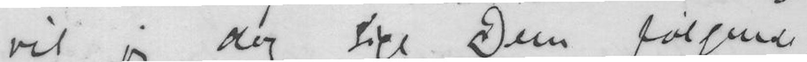vil jeg dog sige Dem følgende,
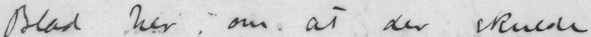Blad her at der skulde
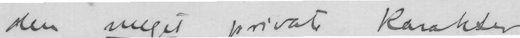den meget private karakter
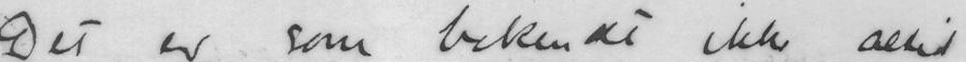Det er som bekendt ikke altid
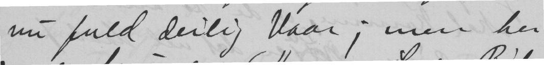nu fuld deilig Vaar; men her
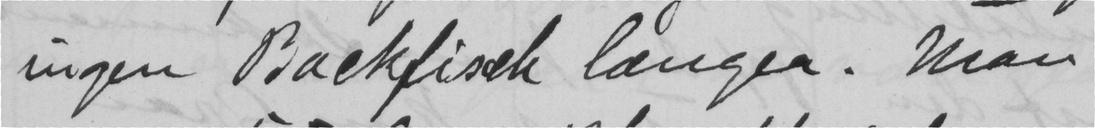ingen Backfisch længer. Man
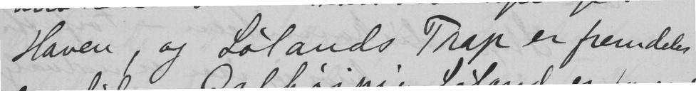Haven, og Lølands Trap er fremdeles
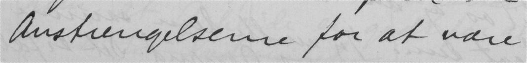Anstrengelserne for at være
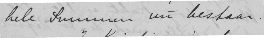hele Summen nu bestaar.
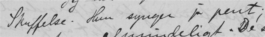Skuffelse. Hun synger jo pent;
